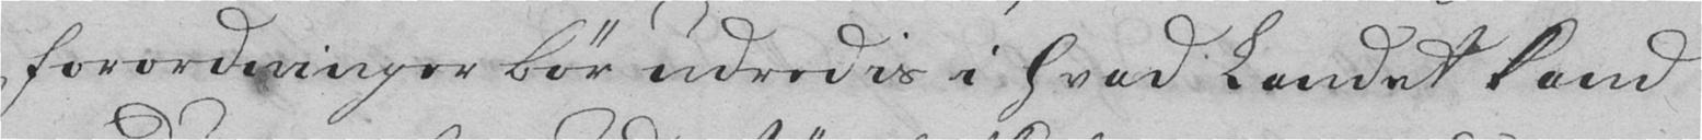forordninger bør udredis i hvad Landet kand
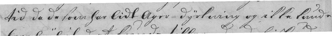tid da de som har lidt Ager-dyrkning og ikke kunde
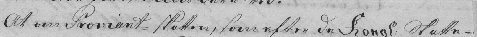At om Proviant-skatten, som efter de Kongl. Skatte-
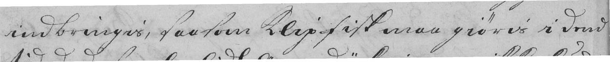indbringis, saasom Klipfisk maa giøris i dend
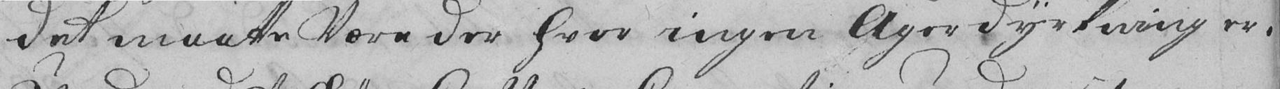det maatte Være der hvor ingen Agerdyrkning er.
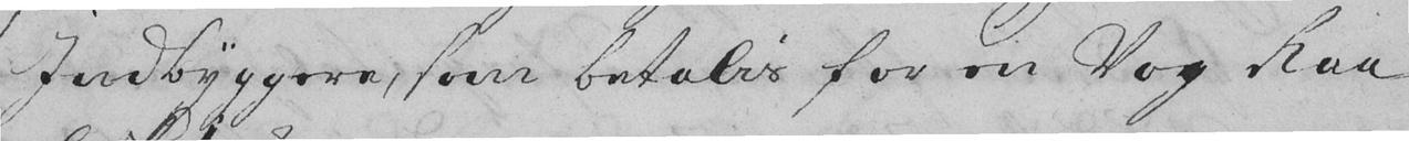Indbyggere, som betalis for en Vog Raa
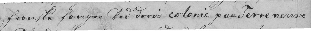Franske fanger ved deris colonie paa Terre neuve
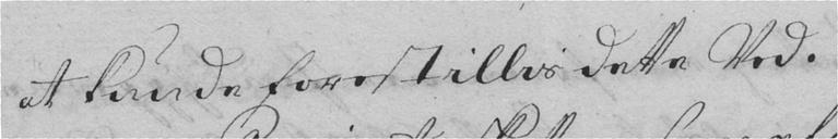at kunde forestillis dette Ved.
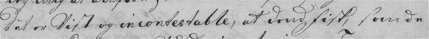Det er Vist og incontestable, at dend Fisk, som de
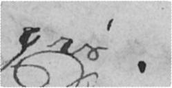gis.
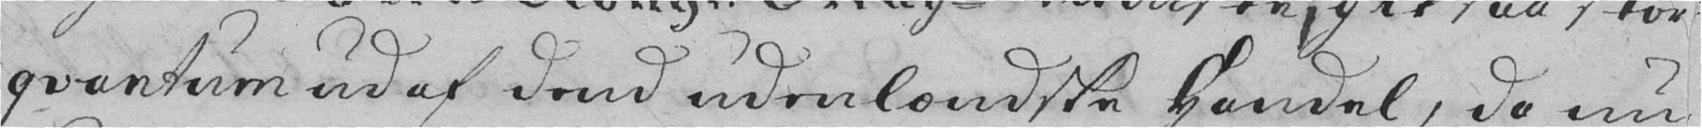qvantum ud af dend udenlandske Handel, da nu
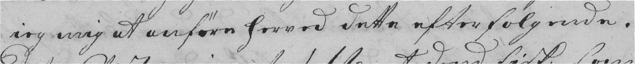ieg mig at anføre herved dette efterfølgende.
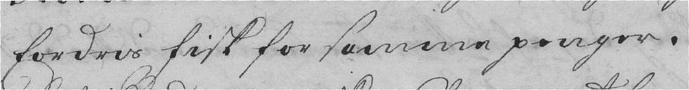fordris Fisk for samme penger.
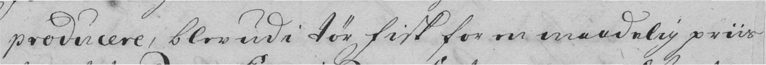producere, blev udi tør Fisk for en maadelig priis
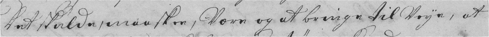Det skulde, maaskee, være og at bringe til Veye, at
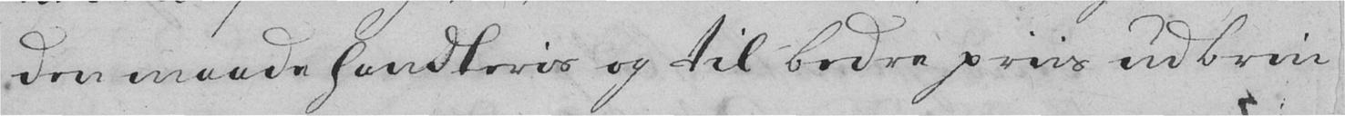den maade handteris og til bedre priis udbrin-
